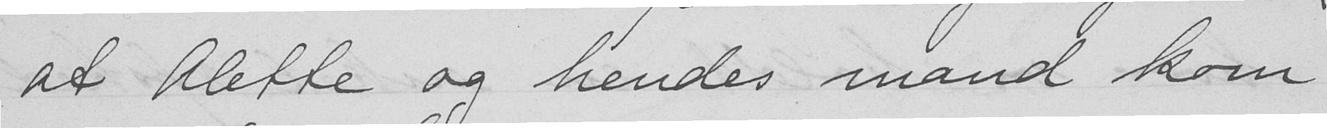at Alette og hendes mand kom
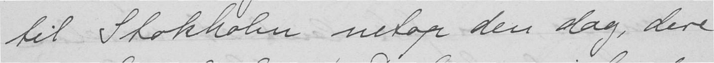til Stokholm netop den dag, dere
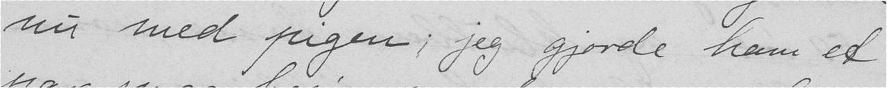nu med pigen; jeg gjorde ham et
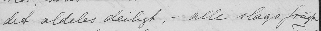det aldeles deiligt, - alle slags frugt-
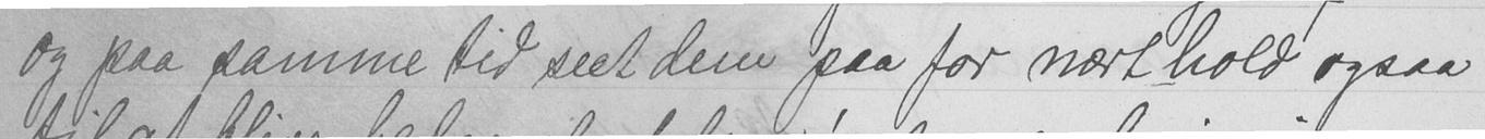og paa samme tid seet dem paa for nært hold ogsaa
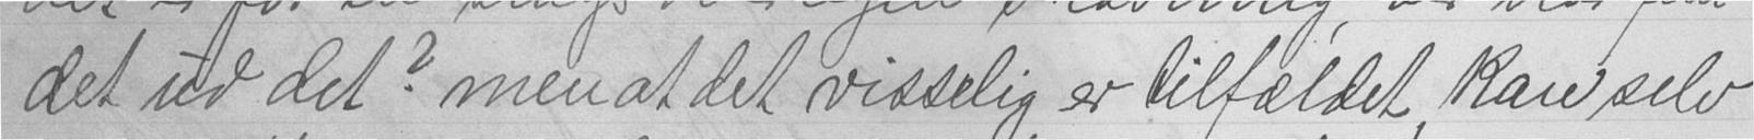det ud det? men at det visselig er tilfældet, kan selv
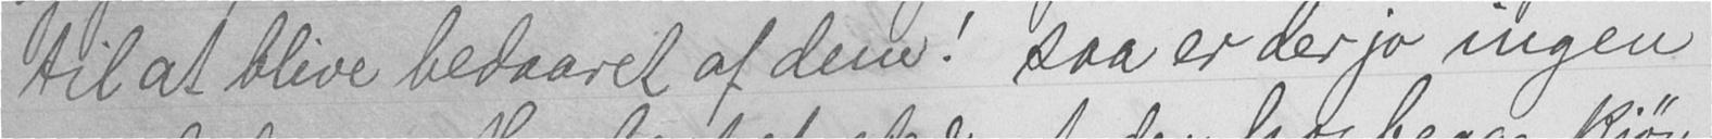til at blive bedaaret af dem! saa er der jo ingen
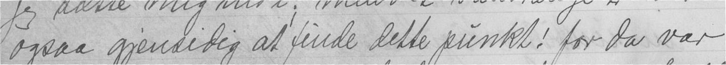ogsaa gjensidig at finde dette punkt! for da var
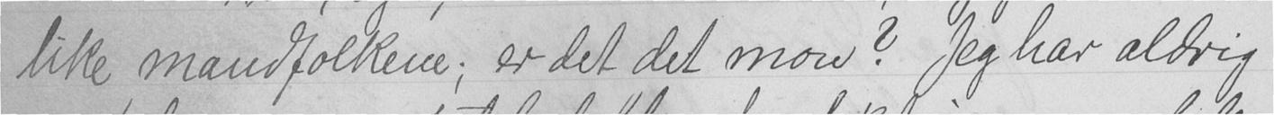like mandfolkene; er det det mon? Jeg har aldrig
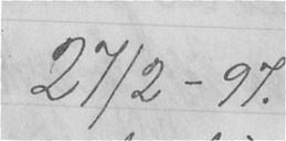27/2-97.
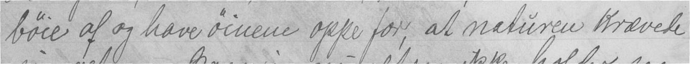bøie af og have øinene oppe for, at naturen krævede
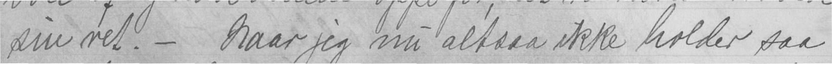sin ret. - Naar jeg nu altsaa ikke holder saa
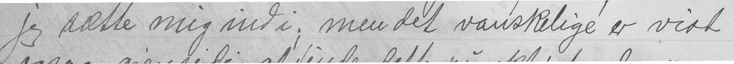jeg sætte mig ind i; men det vanskelige er vist
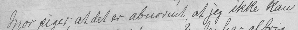Mor siger, at det er abnormt, at jeg ikke kan
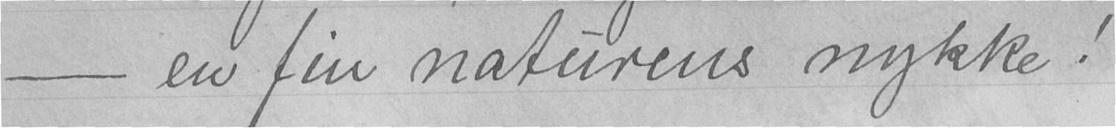- en fin naturens nykke!
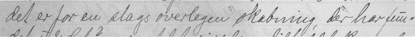det er for en slags overlegen skabning, der har fun-
